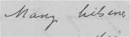Mange hilsener
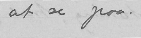at se paa.
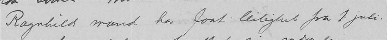Ragnhilds mand har faat leilighet fra 1. juli.
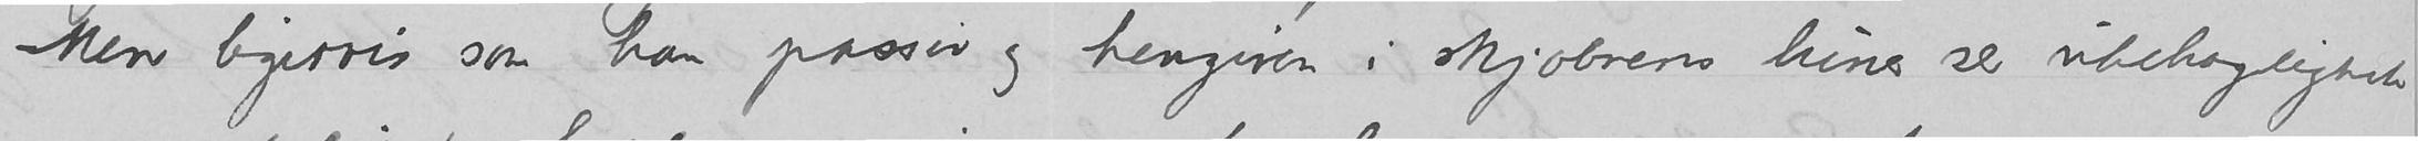Men ligervis som han passer og hengiven i skjobnens hiner ser ubehagelighet
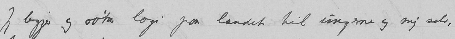Jeg ligger og søker logi paa landet til ungerne og mig selv,
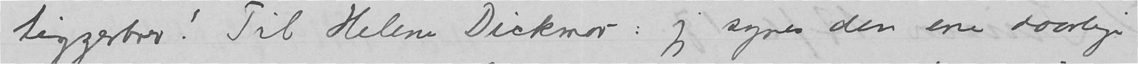tiggerbrev! Til Helene Dickmar : jeg synes den ene daarlige
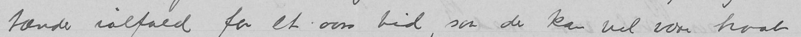tænder ialfald for et aars tid, saa der kan vel være haab
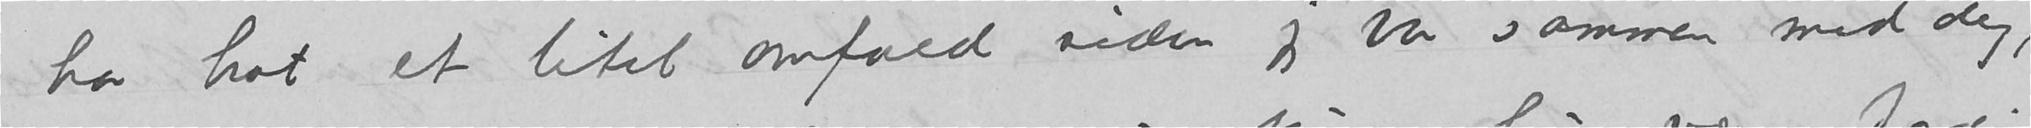har hat et litet anfald siden jeg var sammen med dig,
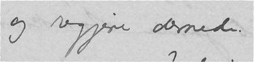og regjere dernede.
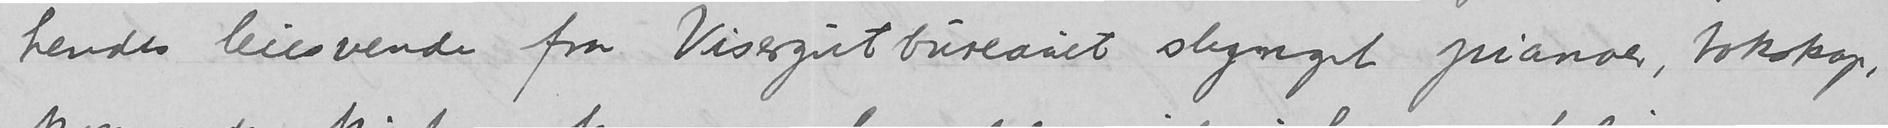hendes leiesvende fra Visergut-bureauet slynget pianoer, tokskap,
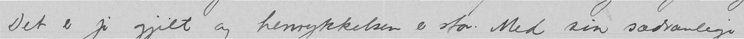Det er jo gjilt og henrykkelsen er stor. Med sin sædvanlige
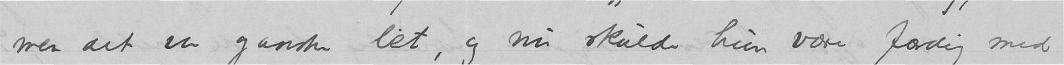men det var ganske let, og nu skulde hun være færdig med
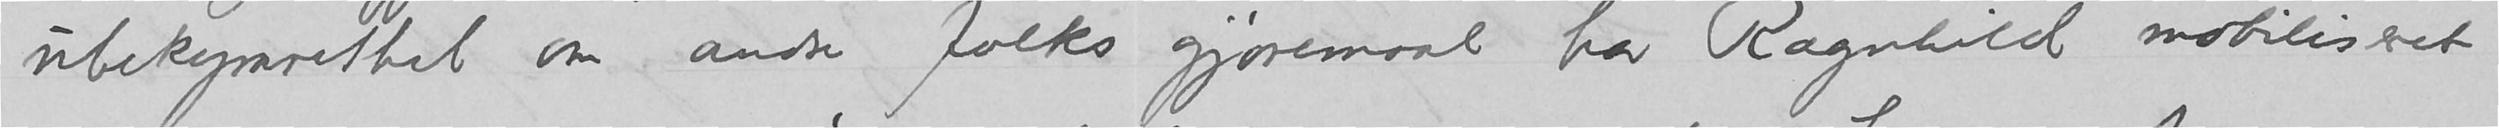ubekymrethet om andre folks gjøremaal har Ragnhild mobiliseret
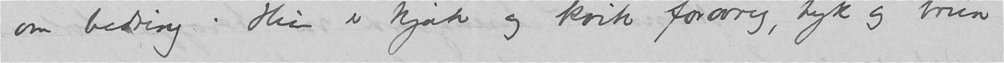om bedring. Hun er kjæk og kvik forøvrig, tyk og brun
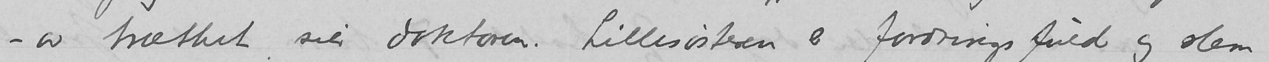av træthet sier doktoren. Lillesøsteren er fordringsfuld og slem
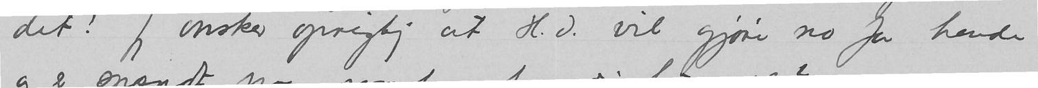det! Jeg ønsker oprigtig at H.D. vil gjøre no for hende
